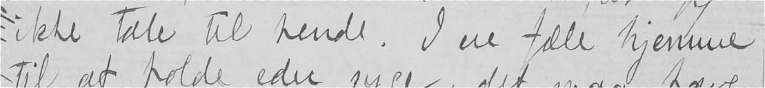ikke tale til hende. I ere fæle hjemme
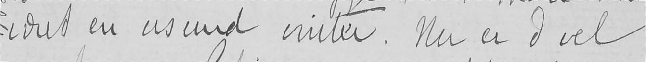været en usund vinter. Nu er I vel
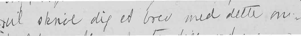vil skrive dig et brev med dette om-
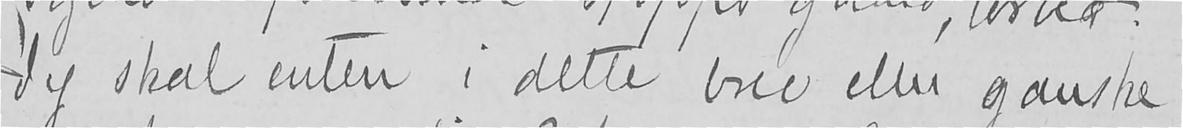Jeg skal enten i dette brev eller ganske
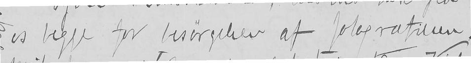os begge for besørgelsen af fotografierne.
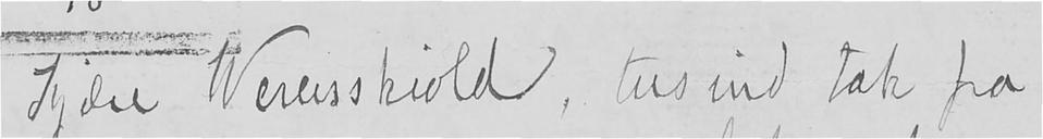Kjære Werenskiold, tusind tak fra
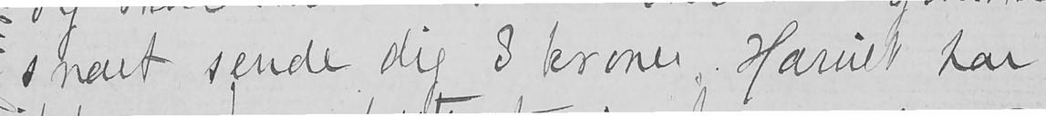snart sende dig 9 kroner. Harriet har
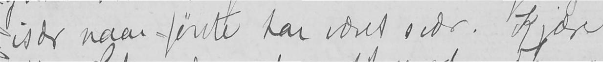især naar første har været svær. Kjære
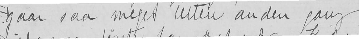gaar saa meget lettere anden gang,
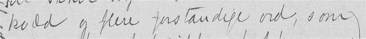kvæd og flere forstandige ord, som
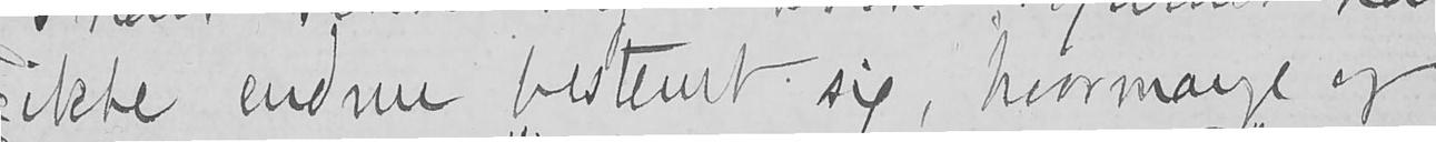ikke endnu bestemt sig, hvormange og
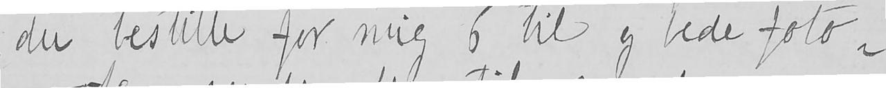du bestille for mig 6 til og bede foto-
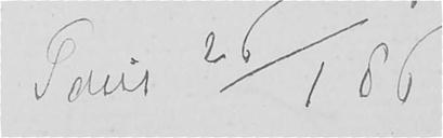Paris 26/1 86
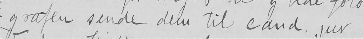grafen sende det til cand. jur
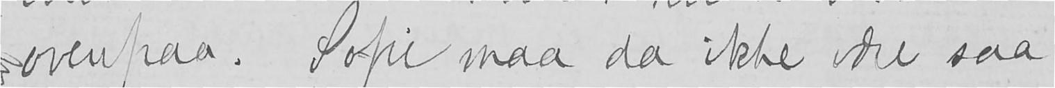ovenpaa. Sofie maa da ikke være saa
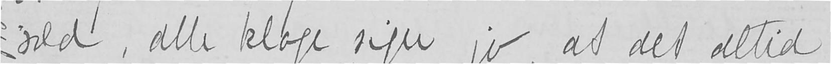ved, alle kloge siger jo, at det altid
